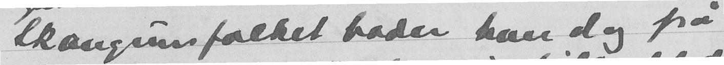Skaugumsfolket bader hver dag fra
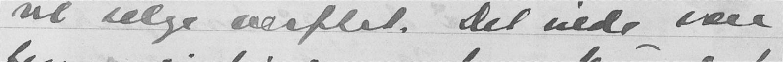vil selge verftet. Det vilde være
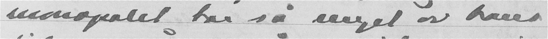monopolet tar så meget av hans
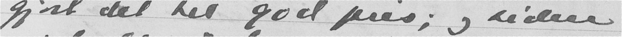gjort det til god pris; og siden
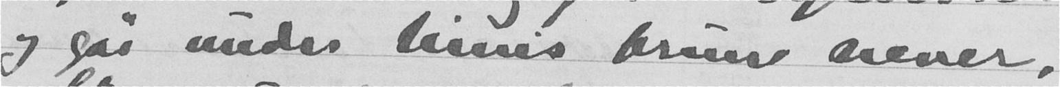og går under leis brune never.
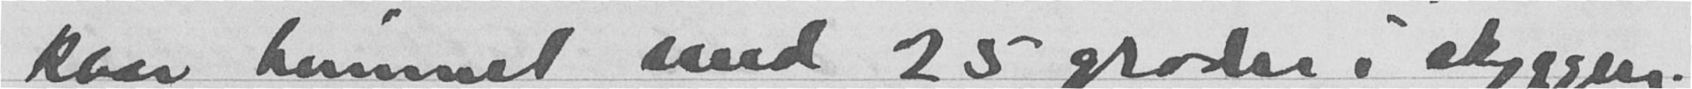klar himmel med 25 grader i skyggen.
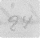94
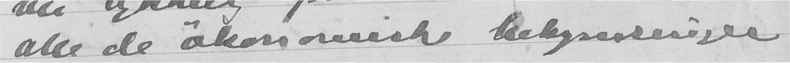alle de økonomiske bekymringer
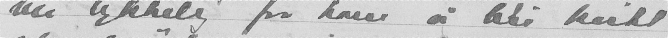bli lykkelig for ham å bli kvitt
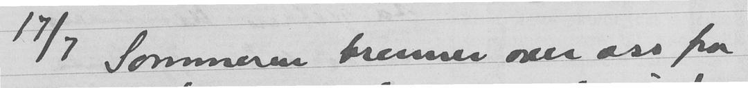17/7 Sommeren brenner over oss fra
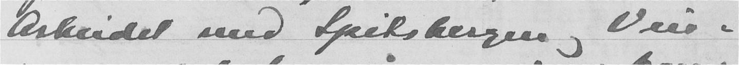Arbeidet med Spitsbergen og Vin-
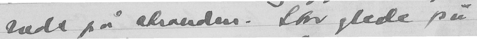nede på stranden. Stor glede på
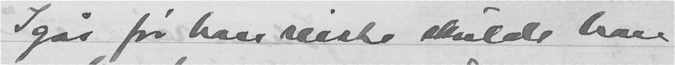Igår før han reiste skulde han
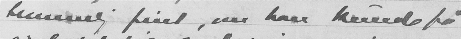temmlig fint, om han kunde få
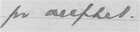for verftet.
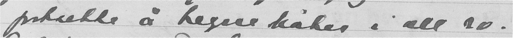fortsette å hegne kåken i all ro.
